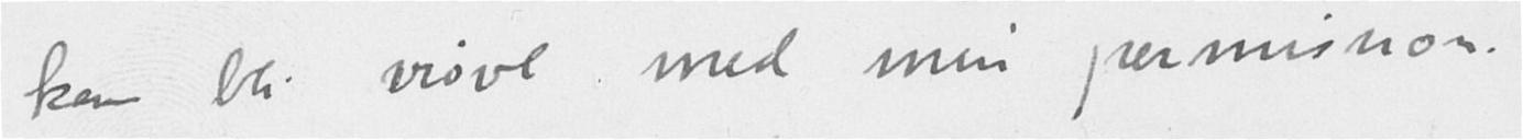kan bli vrøvl med min permission.
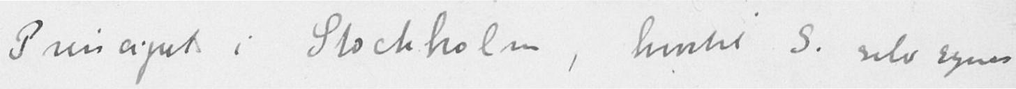Principet i Stockholm, hvortil S. selv synes
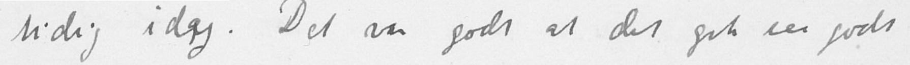tidig i dag. Det var godt at det gik saa godt
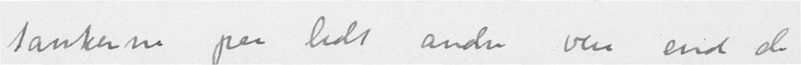tankerne paa lidt andre veie end de
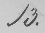13.
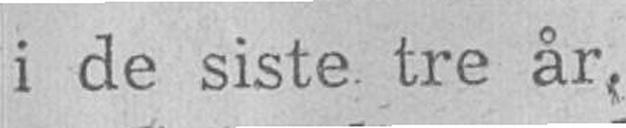i de siste tre år.
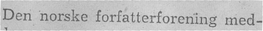Den norske forfatterforening med-
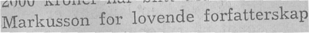Markusson for lovende forfatterskap
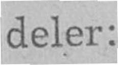deler:
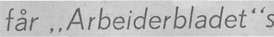får "Arbeiderbladet"s
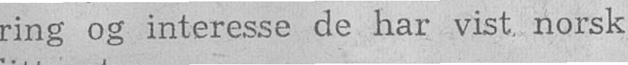ring og interesse de har vist norsk
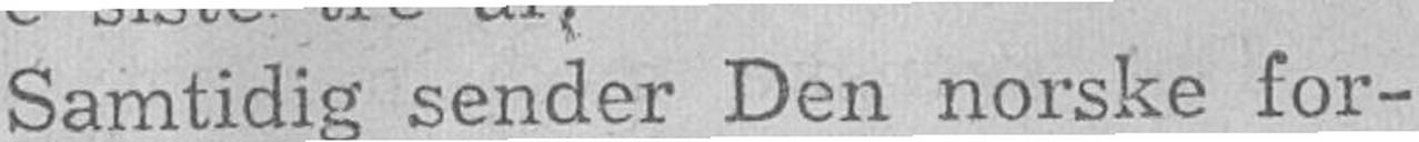Samtidig sender Den norske for-
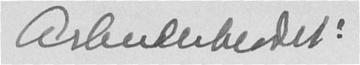Arbeiderbladet:
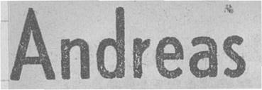Andreas
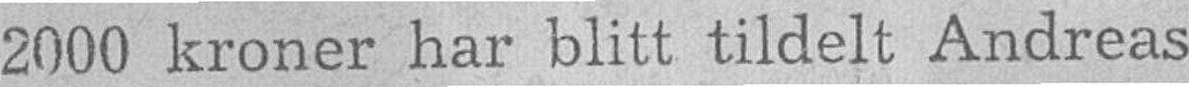2000 kroner har blitt tildelt Andreas
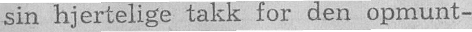sin hjertelige takk for den opmunt-
